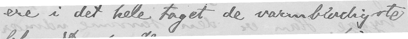ere i det hele taget de varmblodigste
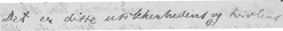Det er disse usikkerhedens og tvivlens
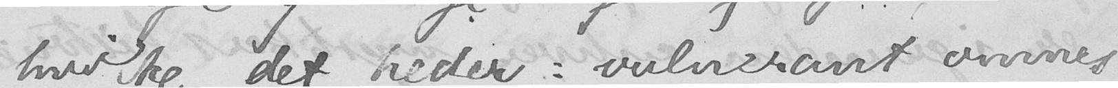hvilke det heder: vulnerant omnes,
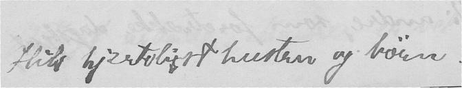Hils hjerteligst hustru og børn.
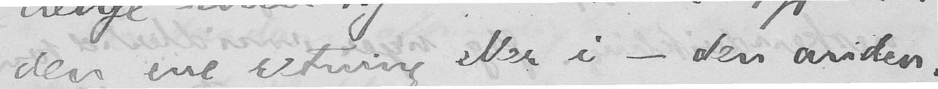den ene retning eller i – den anden.
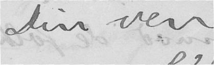Din ven
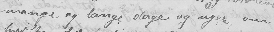mange og lange dage og uger, om
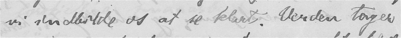vi indbilde os at se klart. Verden tager
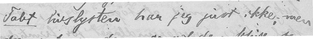Tabt livslysten har jeg just ikke; men
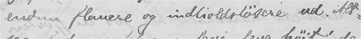endnu flauere og indholdsløsere ud. Alt-
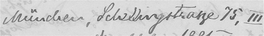München, Schellingstrasse 75, III.
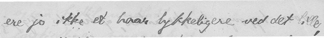ere jo ikke et haar lykkeligere ved det lille,
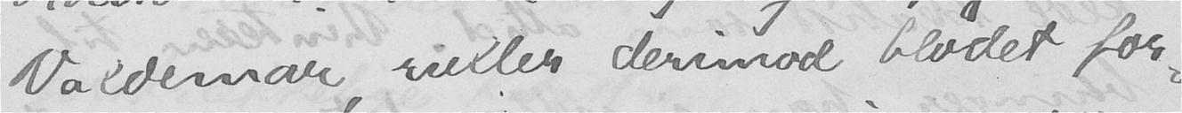Valdemar, ruller derimod blodet for-
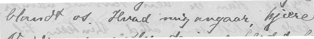blandt os. Hvad mig angaar, kjære
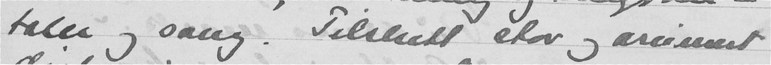taler og sang. Tilslutt stor og ansinnet
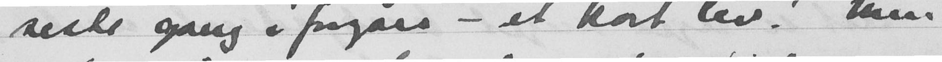siste gang i forgårs - et kort liv. Men
